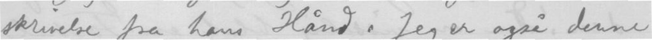skrivelse fra hans Hånd. Jeg er også denne
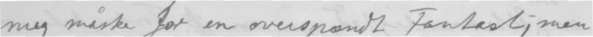mig måske for en overspændt Fantasi, men
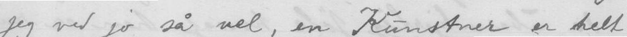jeg ved jo så vel, en Kunstner er helt
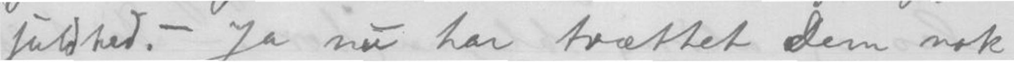fuldhed. - Ja nu har trættet Dem nok,
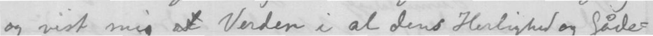og vist mig et Verden i al dens Herlighed og Gåde-
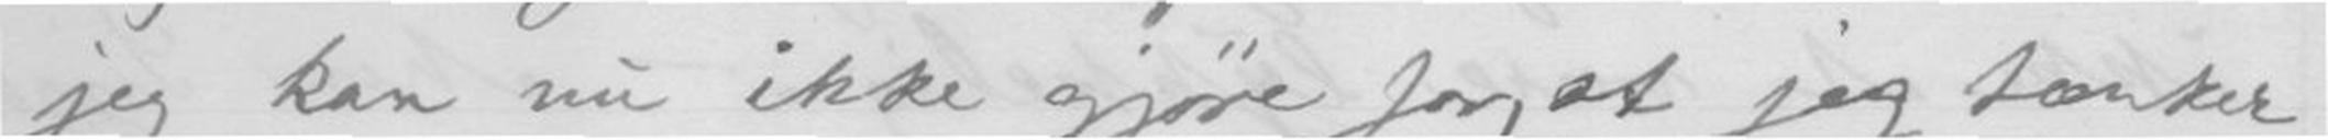jeg kan nu ikke gjøre for, at jeg tænker
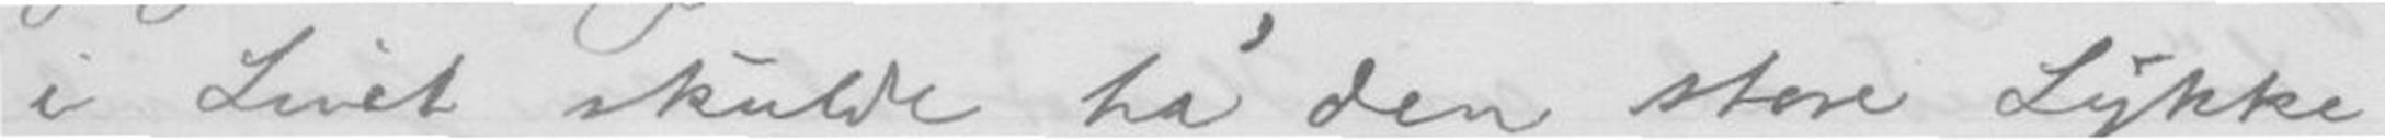i Livet skulde ha' den store Lykke
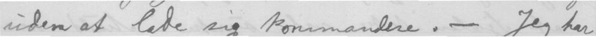uden at lade sig kommandere. - Jeg har
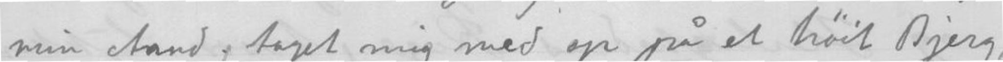min Aand taget mig med op på et høit Bjerg,
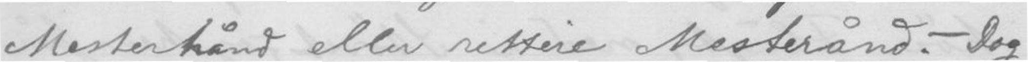Mesterhånd eller rettere Mesterånd.- Dog
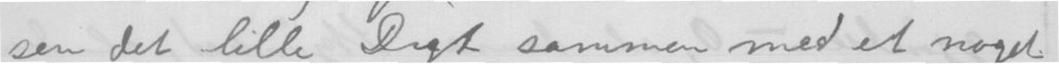sen detlille Digt sammen med et noget
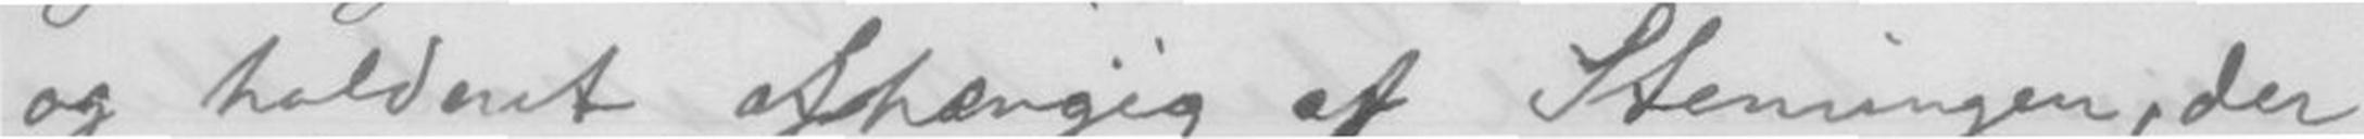og holdent afhængig af Stemingen, der
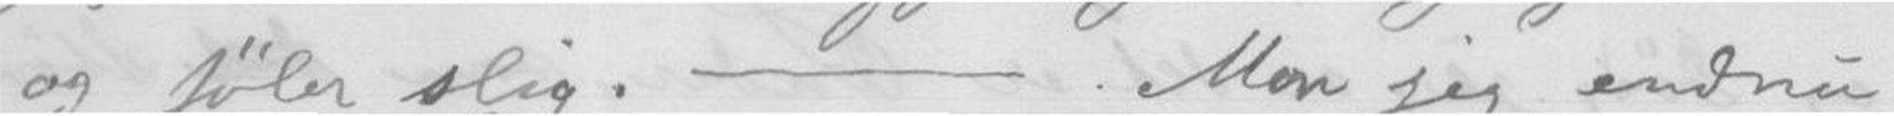og føler slig. - Mon jeg endnu
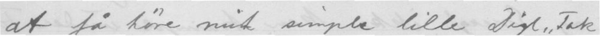at få høre mit simple lille Digt "Tak
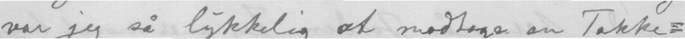var jeg så lykkelig at modtage en Takke-
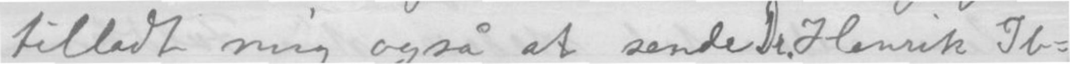tilladt mig også at sende Dr. Henrik Ib-
